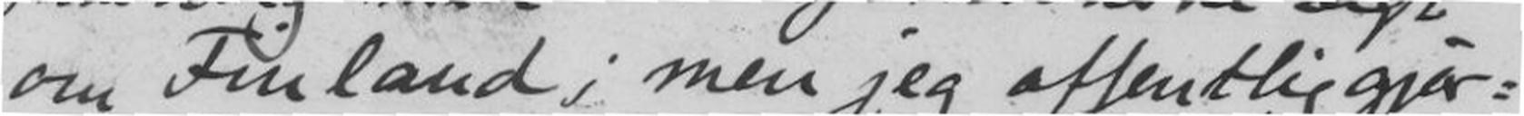om Finland; men jeg offentliggjor=
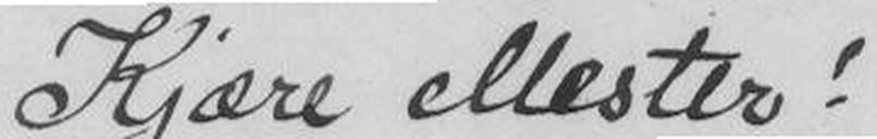Kjære Mester!
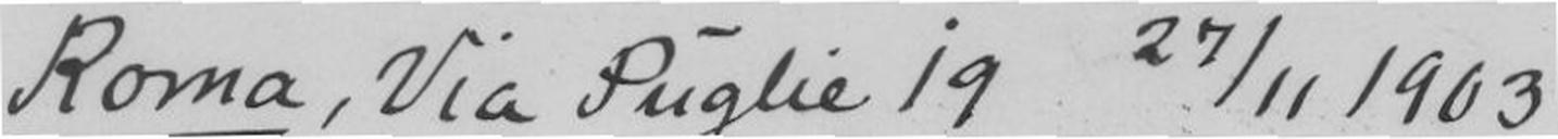Roma, Via Puglie 1927/11 1903
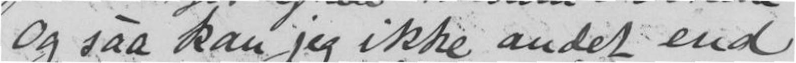Og saa kan jeg ikke andet end
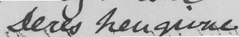Deres hengivne
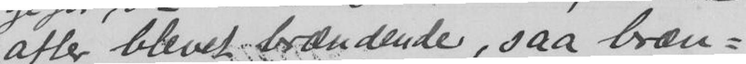atter blevet brændende, saa bræn=
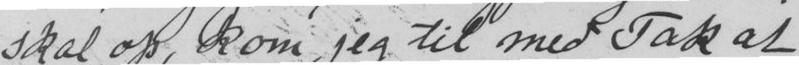skal op, kom jeg til med Tak at
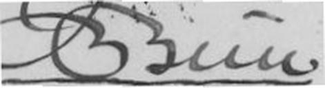JBBull
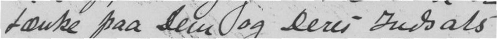tænke paa Dem og Deres Indsats
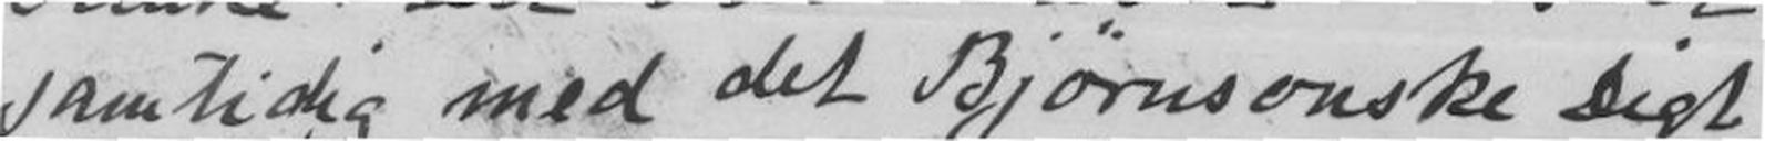samtidig med det Bjørnsonske Digt
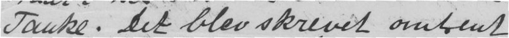Tanke. Det blev skrevet omtrent
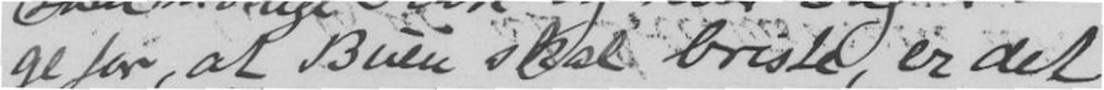ge for, at Buen skal briste, er det
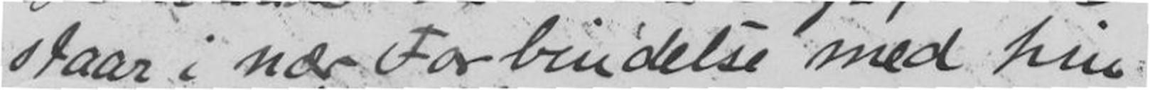staar i nær Forbindelse med hin
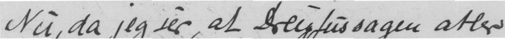Nu, da jeg ser, at Dreyfussagen atter
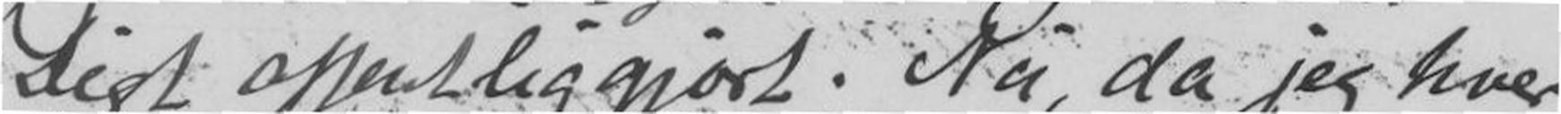Digt offentliggjort. Nu, da jeg hver
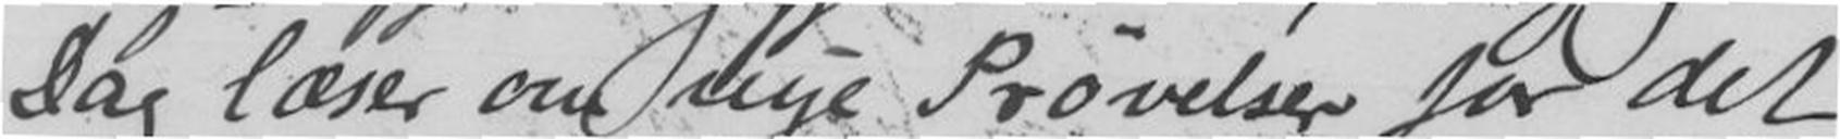Dag læser om nye Prøvelser for det
